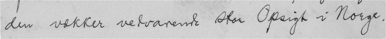den vækker vedvarende stor Opsigt i Norge.
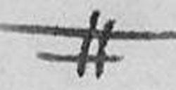#
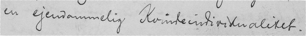en ejendommelig Kvindeindividualitet.
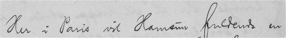Her i Paris vil Hamsun fuldende en
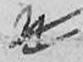#
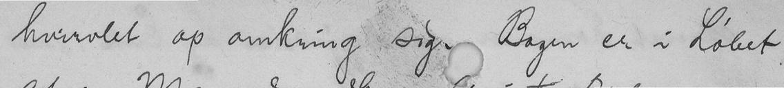hvirvlet op omkring sig. Bogen er i Løbet
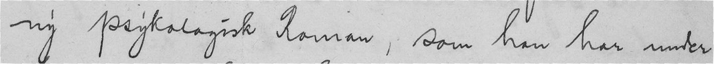ny Psykologisk Roman, som han har under
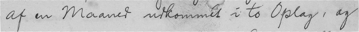af en Maaned udkommet i to Oplag, og
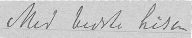Med bedste hilsen
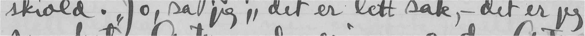skiold. "Jo, sa jeg, "det er lett sak, - det er jeg
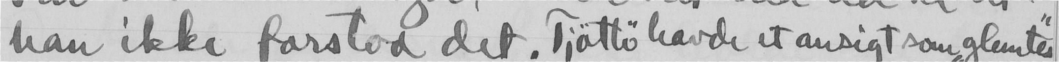han ikke forstod det. Tjøttø havde et ansigt som "glemtes"
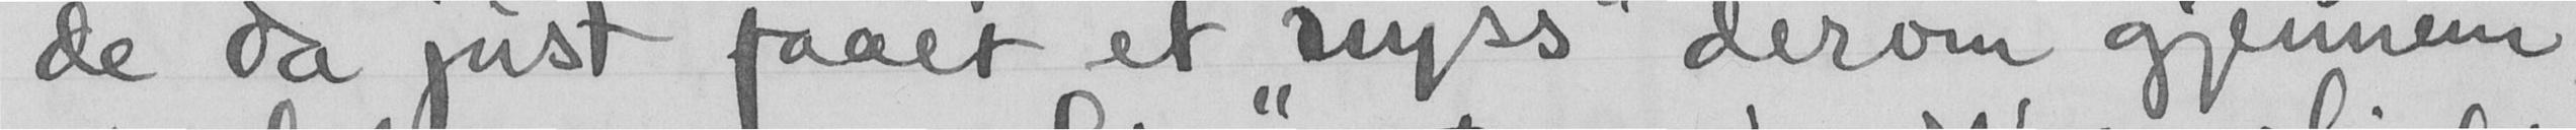de da just faaet et "nyss" derom gjennem
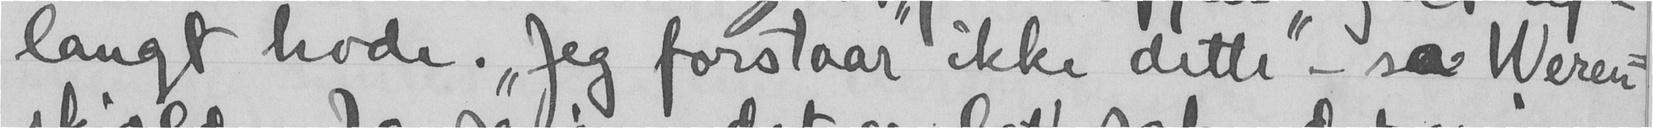langt hode. "Jeg forstaar ikke dette - sa Weren-
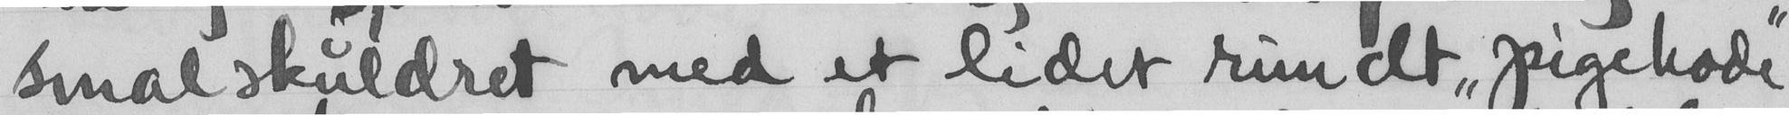smalskuldret med et lidet rundt "pigehode"
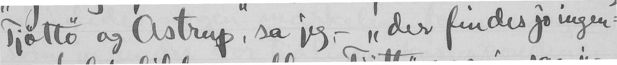Tjøttø og Astrup, sa jeg, - "der findes jo ingen-
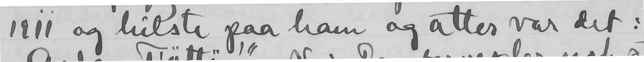1911 og hilste paa ham og atter var det:
